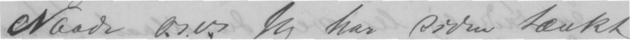Naade o.s.v. Jeg har siden tænkt
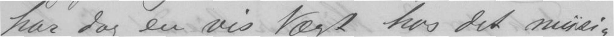har dog en vis Vægt hos det musikalske -
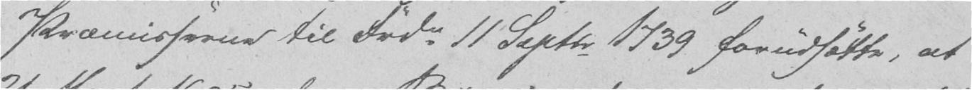Præmisserne til Frdn 11 Septbr. 1739 forudsætte, at
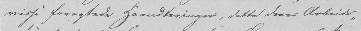visse foragtede Haandteringer, dette deres Arbeide
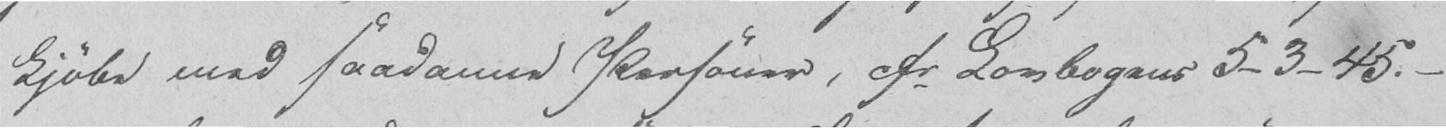kjøbe med saadanne Personer, cfr Lovbogens 5-3-45. -
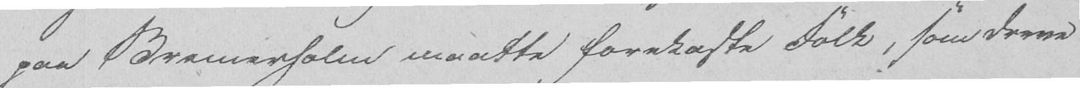paa Bremerholm maatte forekaste Folk, som dreve
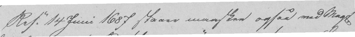Res. 14 Juni 1687 staaer maaskee ogsaa ved Magt.
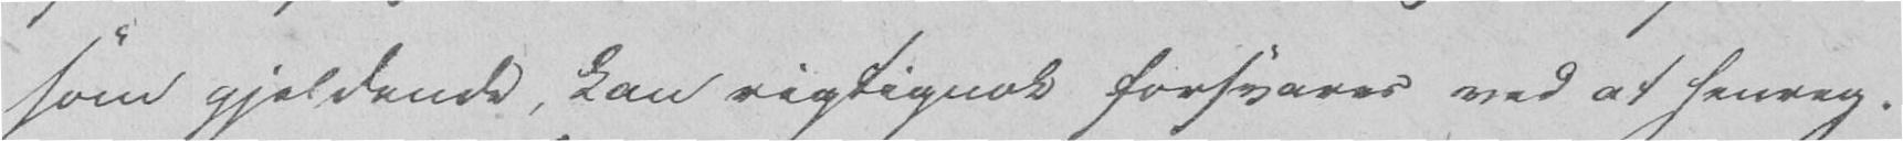som gjeldende, kan rigtignok forsvares ved at henreg-
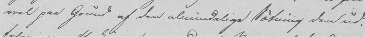vel paa Grund af den almindelige Sætning den ud-
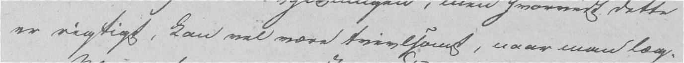er rigtigt, kan vel være tvivlsomt, naar man læg-
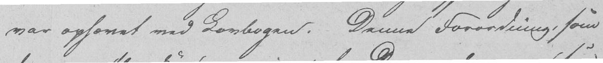var ophævet ved Lovbogen. Denne Forordning, som
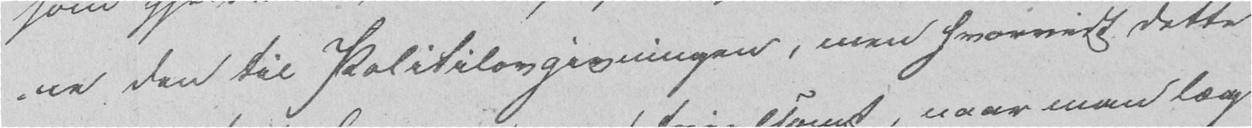ne den til Politilovgivningen, men hvorvidt dette
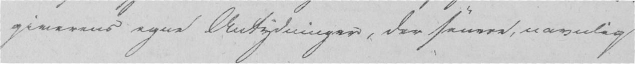giverens egne Antydninger, der senere, navnlig
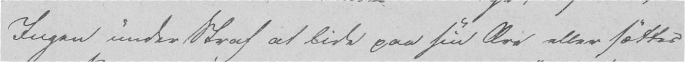Ingen under Straf at lide paa sin Ære eller sættes
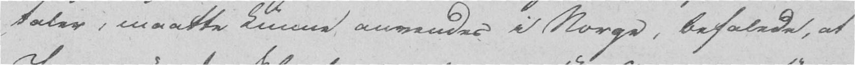taler, maatte kunne anvendes i Norge, befalede, at
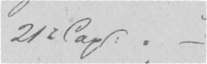21e Cap.. -
